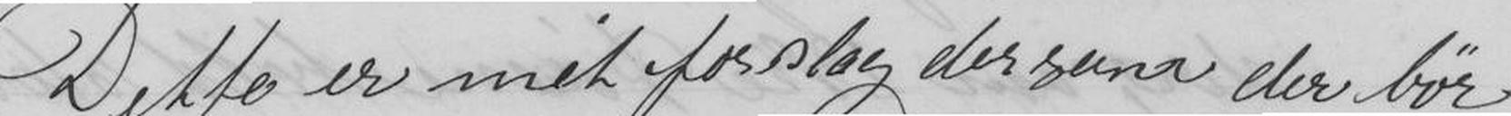Dette er mit forslag dersom der bør
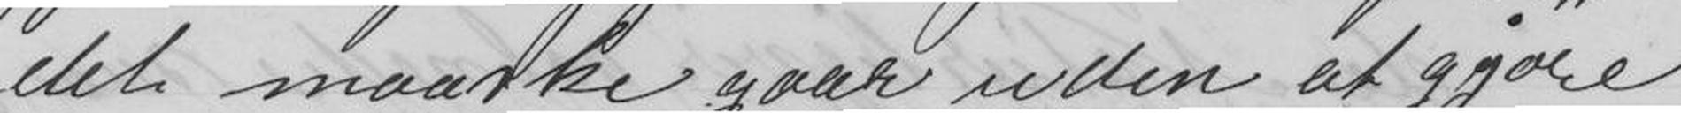det maaske gaar uden at gjøre
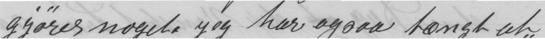gjøres noget. Jeg har ogsaa tængt at
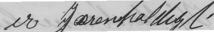er jærnholdigt.
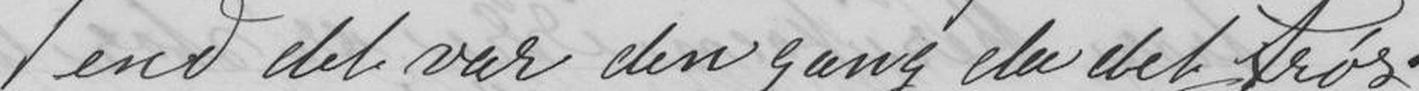end det var den gang da det frøs.
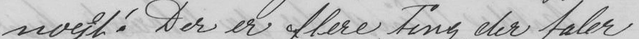noget! Der er flere Ting der taler
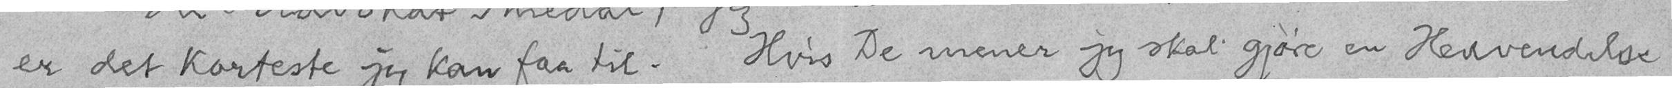er det korteste jeg kan faa til. Hvis De mener jeg skal gjøre en Henvendelse
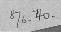8/6. 40.
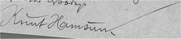Knut Hamsun.
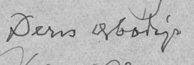Deres ærbødige
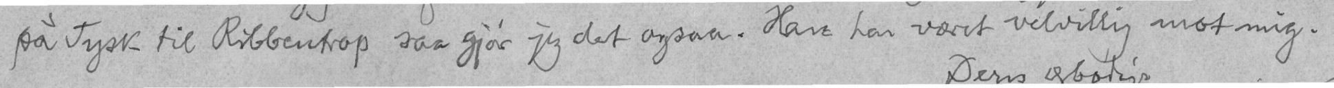på Tysk til Ribbentrop saa gjør jeg det ogsaa. Han har været velvillig mot mig.
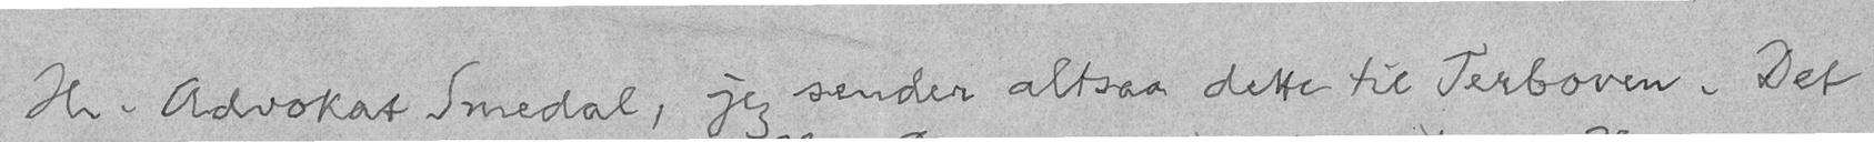Hr. Advokat Smedal, jeg sender altsaa dette til Terboven. Det
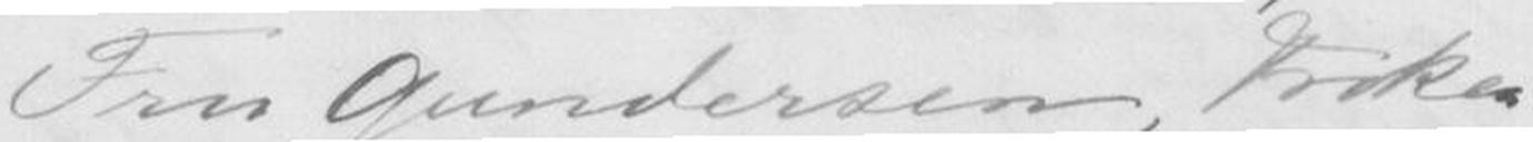Fru Gundersen, Frøken
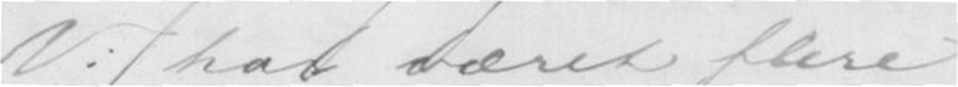Vi har været flere
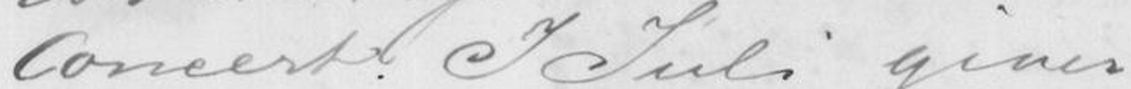Concert. I Juli giver
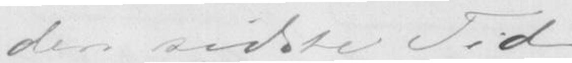den sidste Tid,
